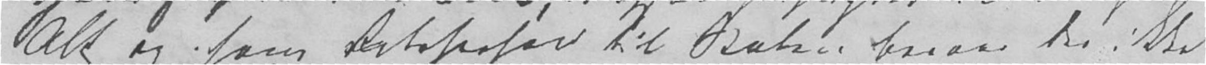Alt og som Retsperson til Staten beroer der ikke
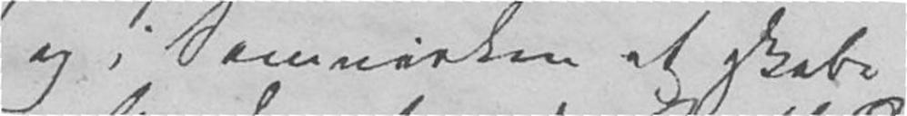og i Samvirken at skabe
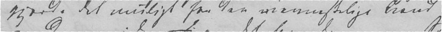gjorde det muligt for den menneskelige Aand
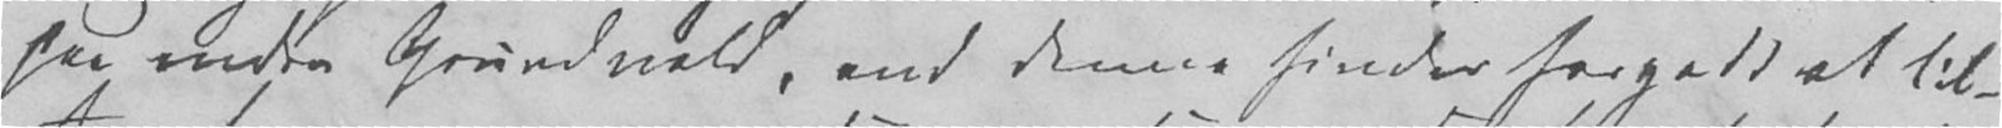paa anden Grundvold, end denne finder forgodt at til-
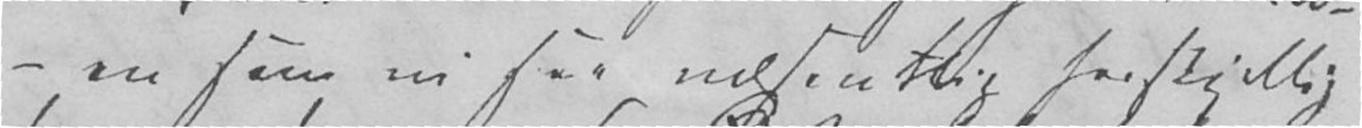– en som vi saa væsentlig forskjellig
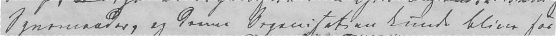Synsmaader, og denne Organisationen kunde blive saa
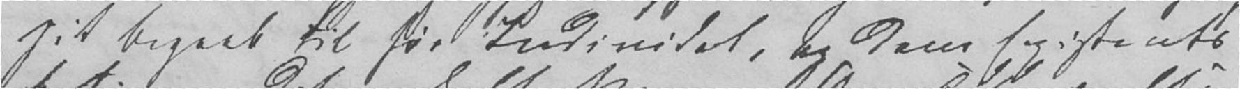sit Begreb til for Individet, og dens Existents
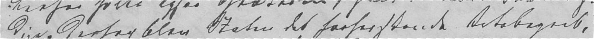dige, Derfor blev Staten det forherskende Retsbegreb,
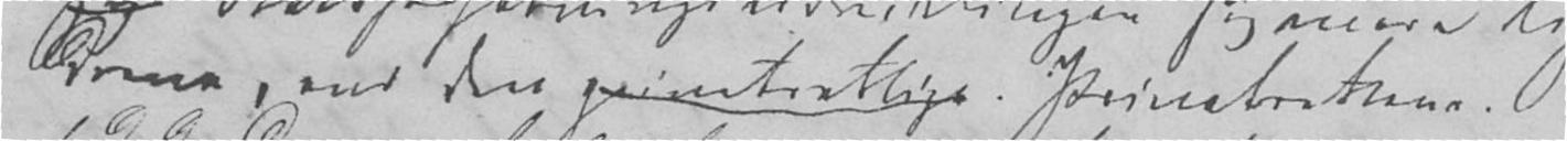derne, end den privatretlige. Privatrettene.
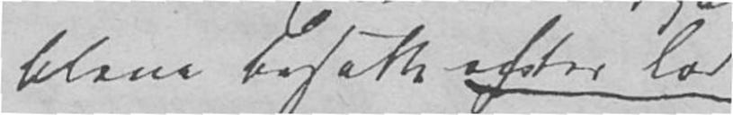bleve besatte efter Lod
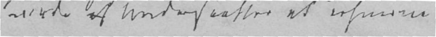mede Undersaatter at erhverve
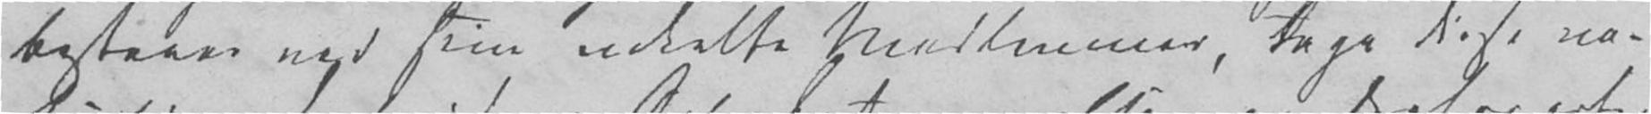bestaaer ved sine enkelte Medlemmer, tage disse na-
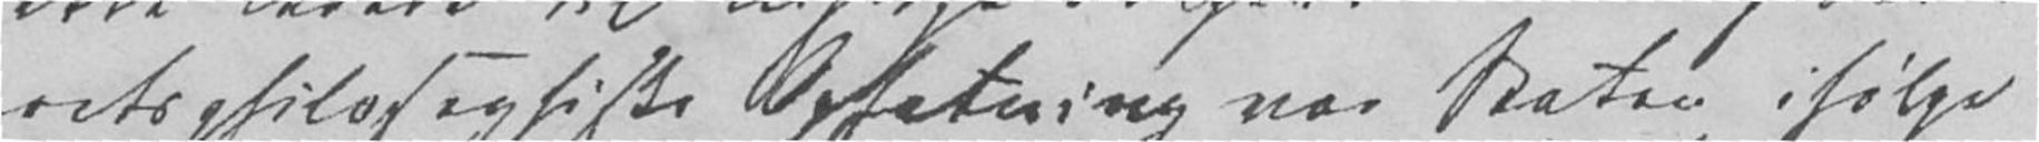retsphilosophiske Opfatning var Staten ifølge
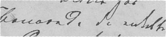bevarede de enkelte
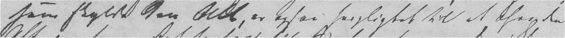som skylde den Alt, er ogsaa forpligtet til at ofre den
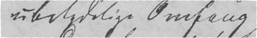ubetydelige Omfang
